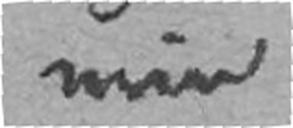min
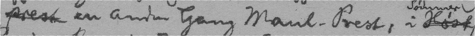prest en anden Gang Maul-Prest, i Høst
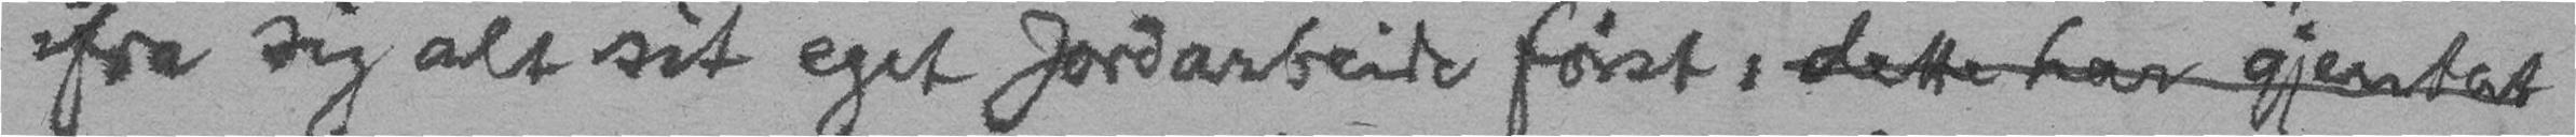ifra sig alt sit eget Jordarbeide først, dette har gjentatt
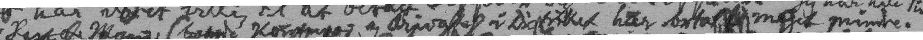Resten i Mars, (baade Korgengag og Brivaled i Distriktet har betalt meget mindre.
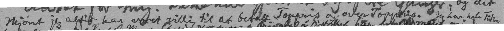skjønt jeg altid har været villig til at betalt Toppris og over Toppris. Jeg har hele Tiden
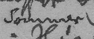Sommer
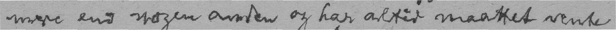mere end nogen anden og har altid maattet vente
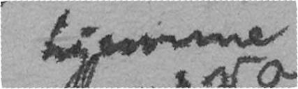hjemme
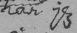har jeg
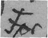For
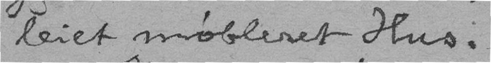leiet møbleret Hus.
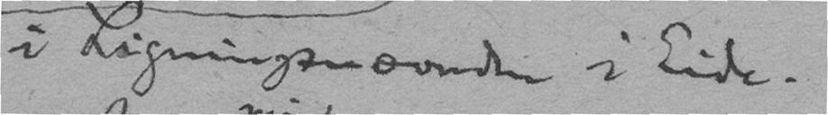i Ligningsnæmden i Eide.
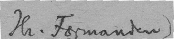Hr. Formanden
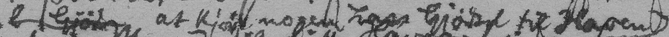b Gjøds at kjøre nogen Lass Gjødsel til Haven)
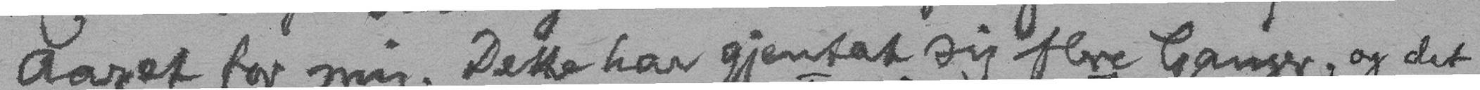Aaret for mig. Dette har gjentat sig flere Ganger, og det
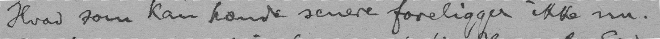Hvad som kan hænde senere foreligger ikke nu.
